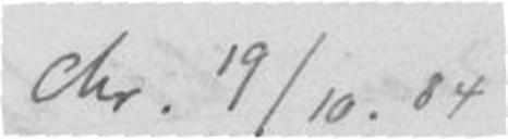Chr. 19/10.84
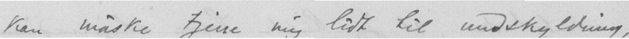kan måske tjene mig lidt til undskyldning,
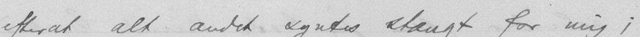efterat alt andet syntes stængt for mig i
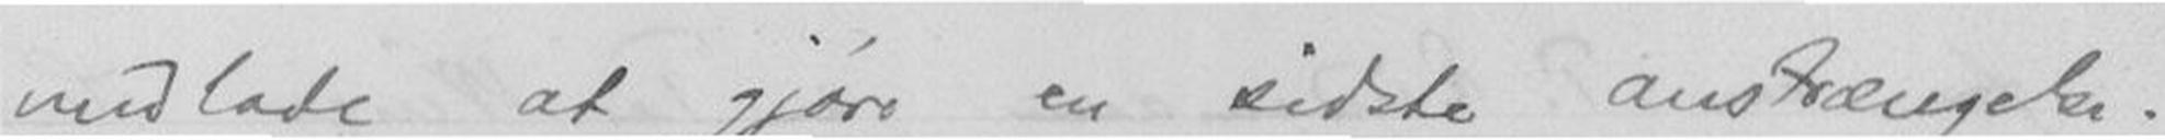nedlade at gjøre en sidste anstrængelse.
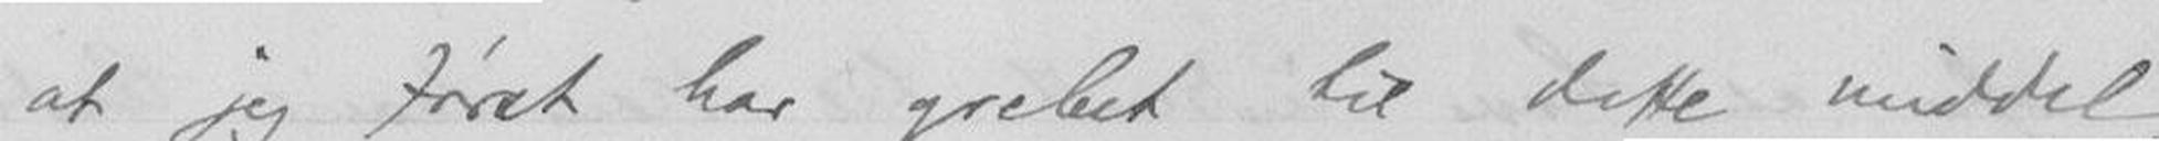at jeg først har grebet til dette middel,
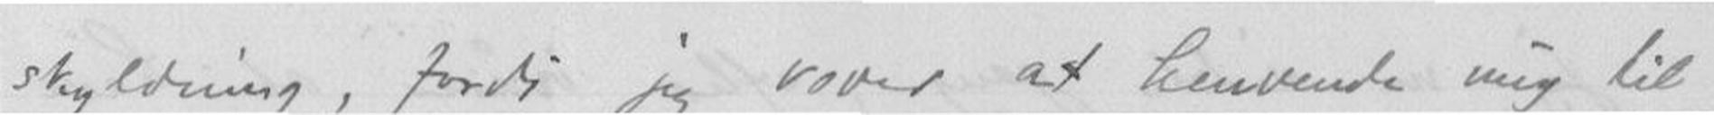skyldning, fordi jeg vover at henvende mig til
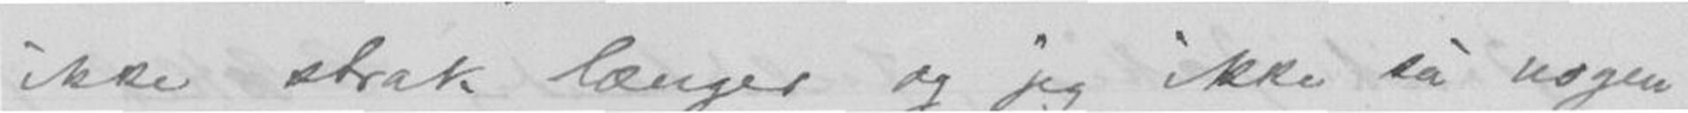ikke strak længer og jeg ikke så nogen
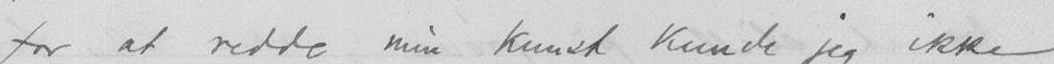for at redde min kunst kunde jeg ikke
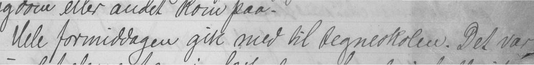Hele formiddagen gik med til tegneskolen. Det var
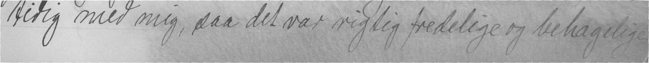tidig med mig, saa det var rigtig fredelige og behagelige
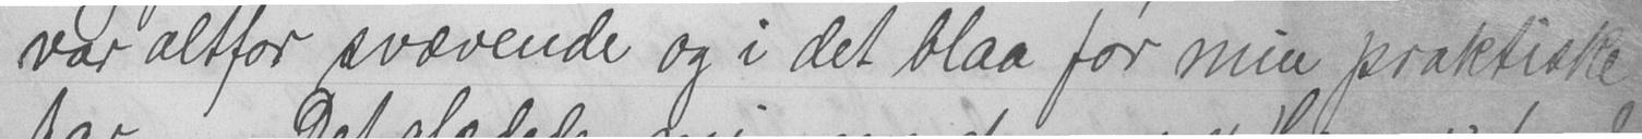var altfor svævende og i det blaa for min praktiske
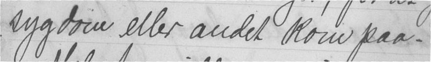sygdom eller andet kom paa.
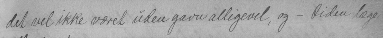det vel ikke været uden gavn alligevel, og - tiden læger
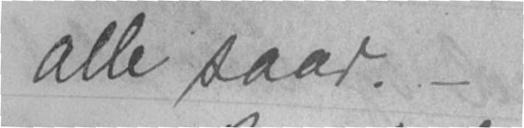alle saar. -
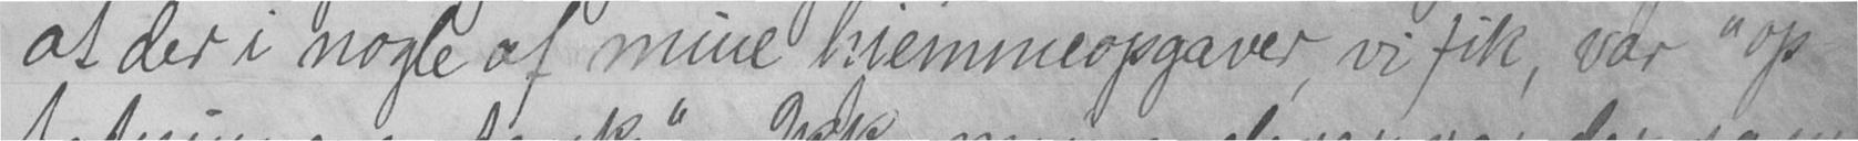at der i nogle af mine hjemmeopgaver, vi fik, var "op-
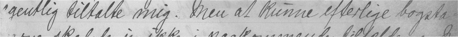egentlig tiltalte mig. Men at kunne efterlige bogsta-
